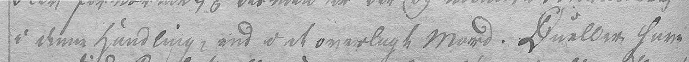i denne Handling, end i et overlagt Mord. Dueller have
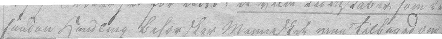saadan Handling behersker Mennesket maa tilbagetræn-
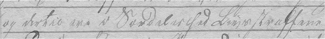og dertil ere i Særdeleshed Livsstraffene
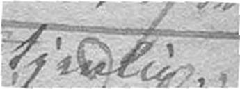tjenlige.
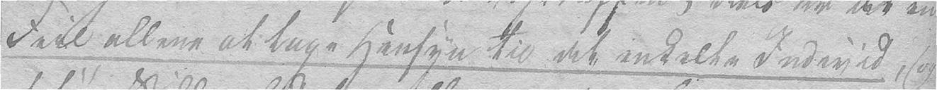Feil allene at tage Hensyn til det enkelte Individ, og
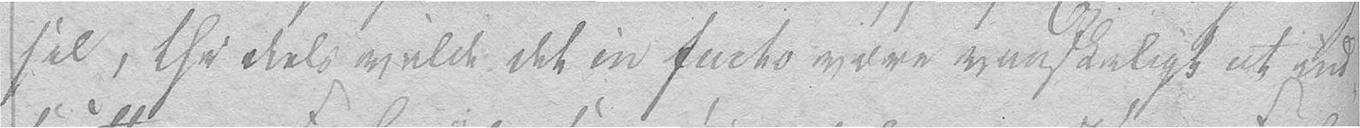sel, thi deels vilde det in facto være vanskeligt at ind-
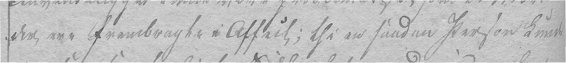der ere frembragte i Affect; thi en saadan Person kunde
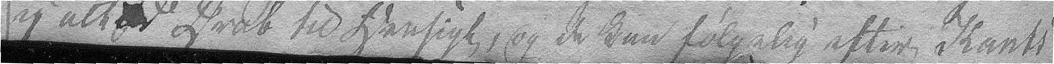ey altid Drab til Hensigt, og de kan følgelig efter Kants
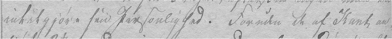intetgjøre sin Personlighed. Foruden de af Kant an-
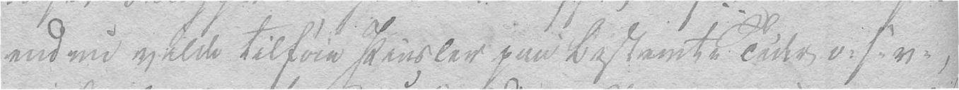enten vilde tilføre Pinsler paa bestemte Tider, o:s:v:,
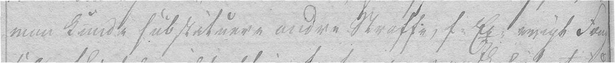man kunde substituere andre Straffe, f:Ex: evigt Fæng-
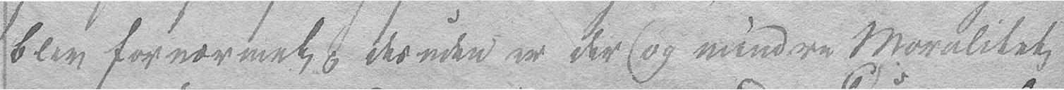blev fornærmet, desuden er der og mindre Moralitet
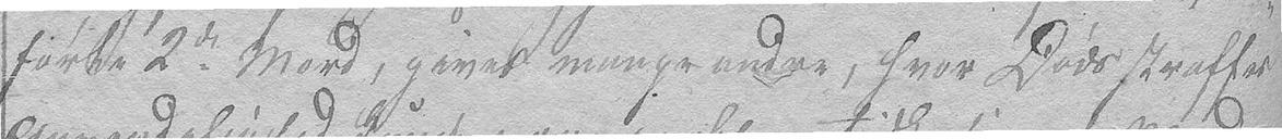førte 2de Mord, gives mange andre, hvor Dødsstraffes
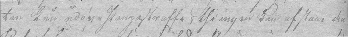ten kun udøve Pengestraffe; thi ingen kan afstaae den
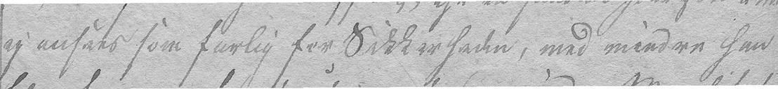ey ansees som farlig for Sikkerheden, med mindre han
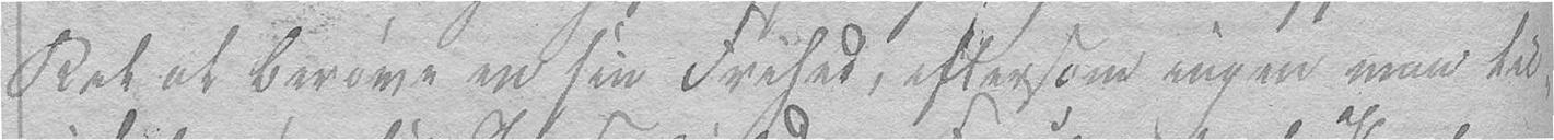Ret at berøve en sin Frihet, eftersom ingen maa til-
